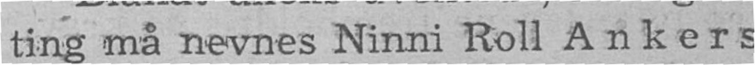ting må nevnes Ninni Roll Ankers
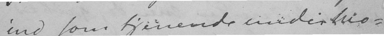ind som tjenende under Mo-
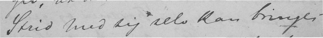Strid med sig selv kan bringes
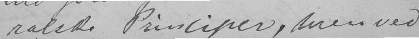ralske Principer, men ved
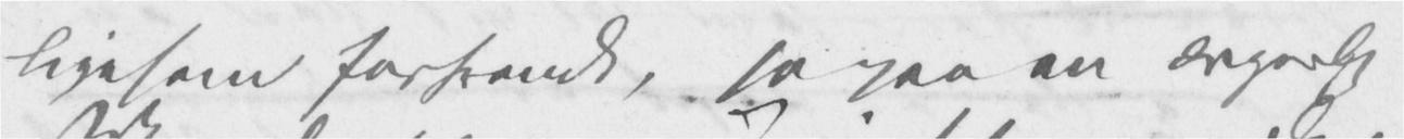ligesom forsvandt, ja paa en ærgerlig
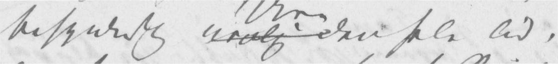besynderlig urolig Uro den hele Tid.
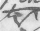<...>
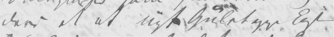drer at et nyt Gulvteppe koster
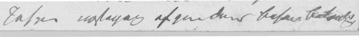Josephine næstegang afgive Deres Besyv Betids
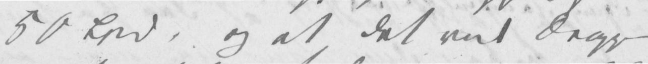50 Spd, og at det Drapperie
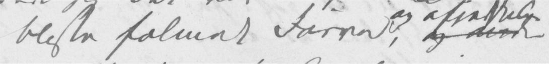blasse falmede Farver, og <...>
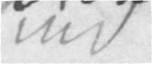ind
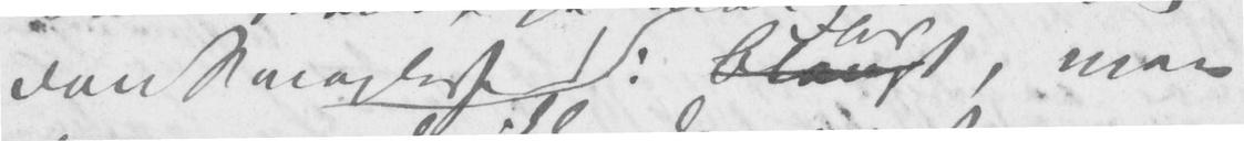den Smagløshed i Blomst, men
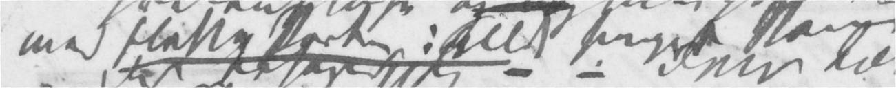der behager sig med fleste Partier
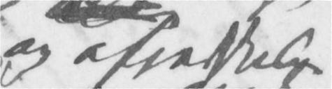og afjadskelige
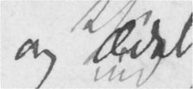og Ædelt
